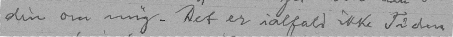din om mig. Det er ialfald ikke Tiden
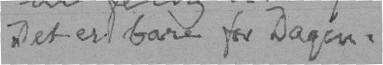Det er bare for Dagen.
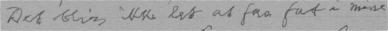Det blir ikke let at faa fat i mine
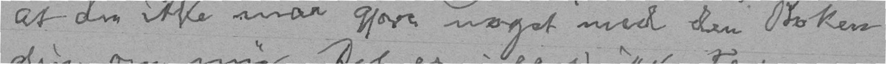at du ikke maa gjøre noget med den Boken
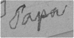Papa
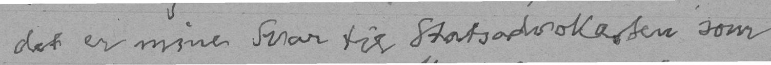det er mine Svar til Statsadvokaten som
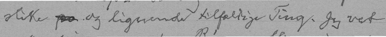slike pa og lignende tilfældige Ting. Jeg vet
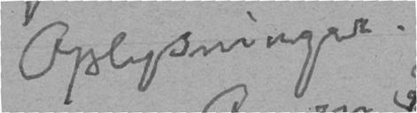Oplysninger.
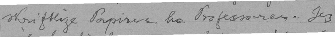skriftlige Papirer hos Professoren. Jeg
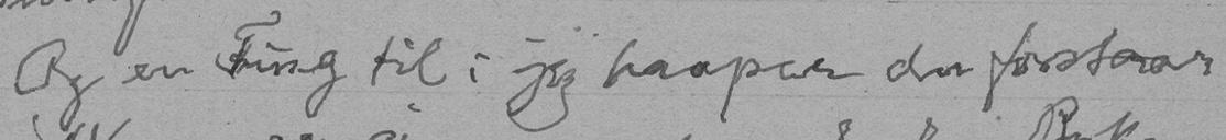Og en Ting til: jeg haaper du forstaar
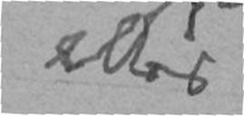eller
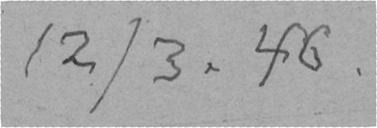12/3. 46.
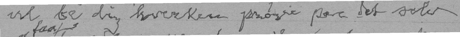vil be dig hverken prøve paa det selv
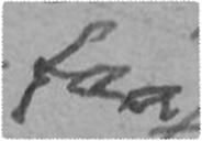faa
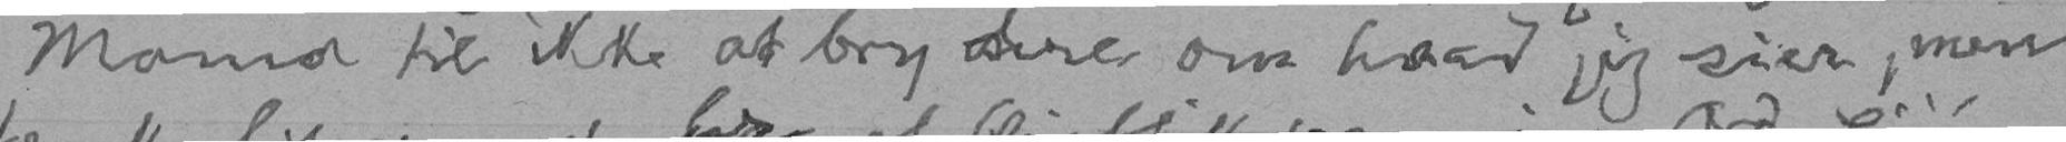Mama til ikke at bry dere om hvad jeg sier, men
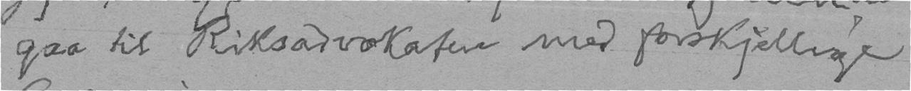gaa til Riksadvokaten med forskjellige
